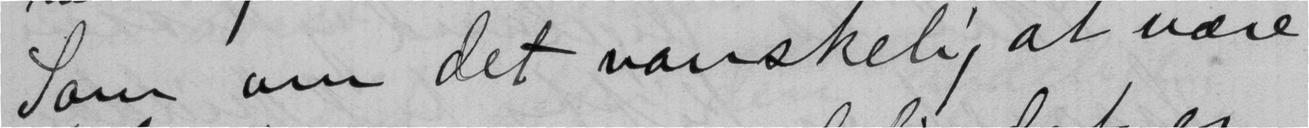Som om det vanskelig at være
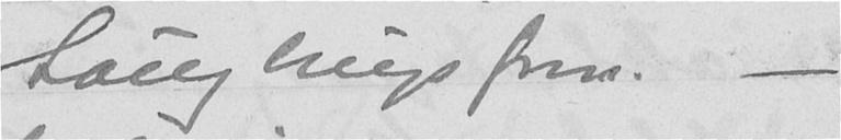Saugbrugsforn. -
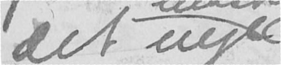det nye
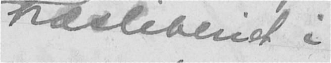træsliberiet i
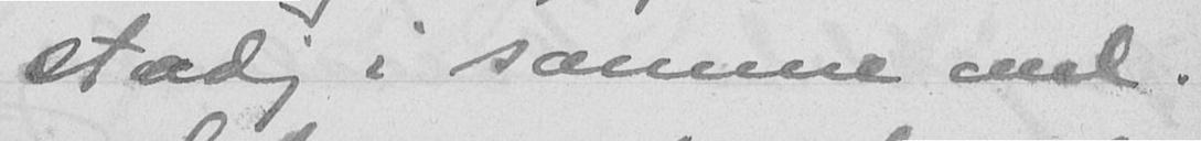Stadig i samme anl.
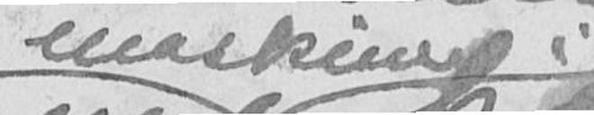maskinen i
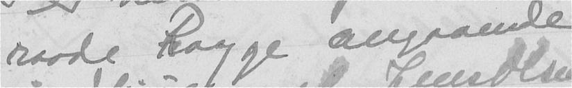raade Rogge angaaende
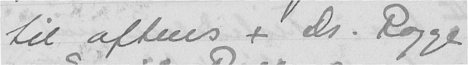til aftens + Dr. Rogge
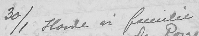30/1 Havde vi familie
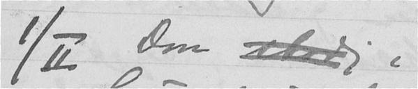1/II Don stadig i
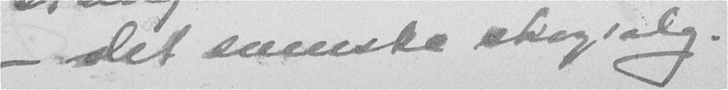- det svenske skogsalg.
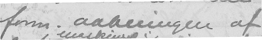form. aabningen af
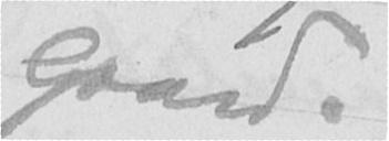Gaard.
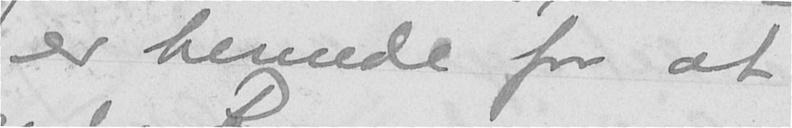er hernede for at
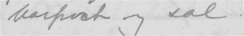barfrost og sol.
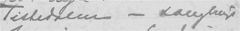Tistedalen - Saugbrugs
